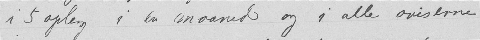i 5 oplag i en maaned og i alle aviserne
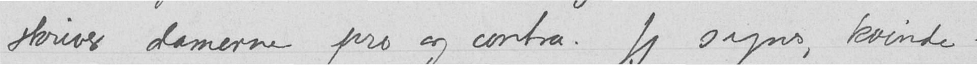skriver damerne pro og contra. Jeg synes, kvinde-
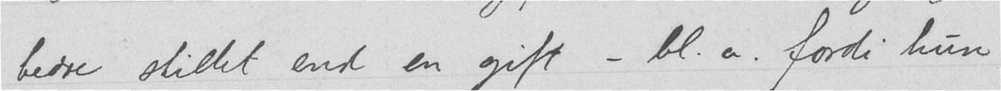bedre stillet end en gift - bl.a. fordi hun
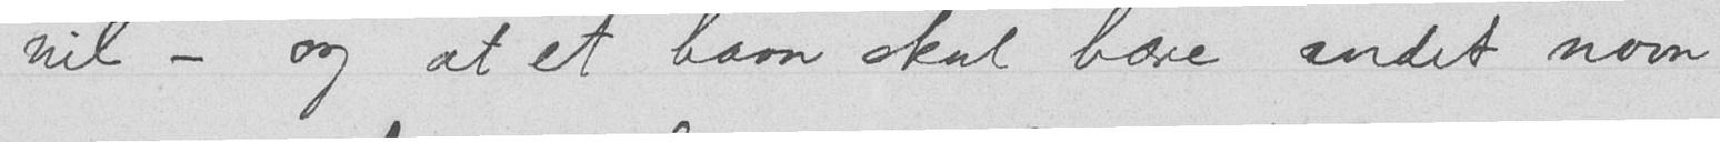vil - og at et barn skal bære andet navn
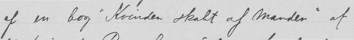af en bog "Kvinden skabt af manden" af
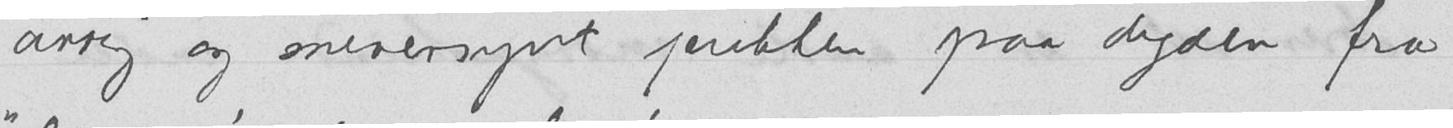arrig og sneversynt pukken paa dyden fra
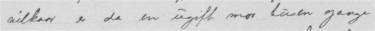vilkaar er da en ugift mor tusen gange
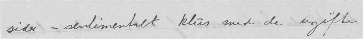sider - sentimentalt klus med de ugifte
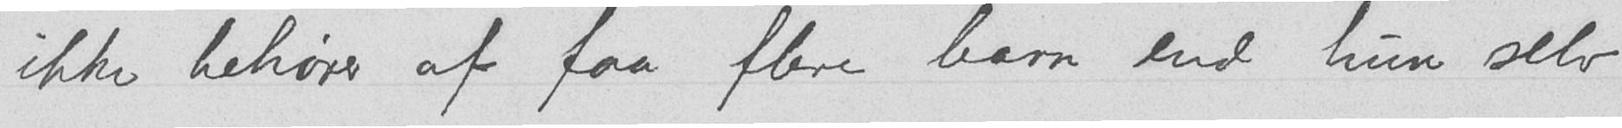ikke behøver at faa flere barn end hun selv
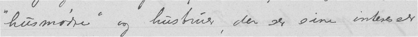"husmødre" og hustruer, der ser sine interesser
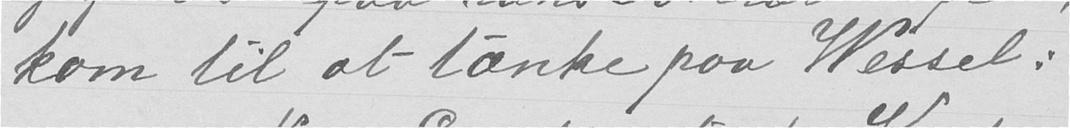kom til at tænke paa Wessel:
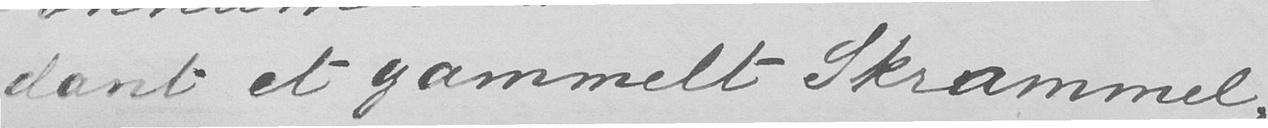dant et gammelt Skrammel,
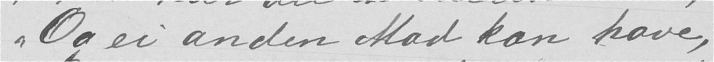"Og ei anden Mad kan have,
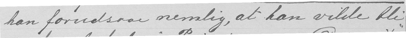han forudsaae nemlig, at han vilde bli-
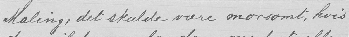Maling, det skulde være morsomt, hvis
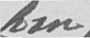han
